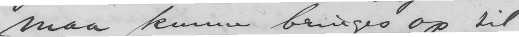maa kunne bringes op til
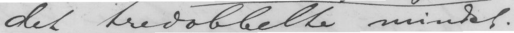det tredobbelte mindst.
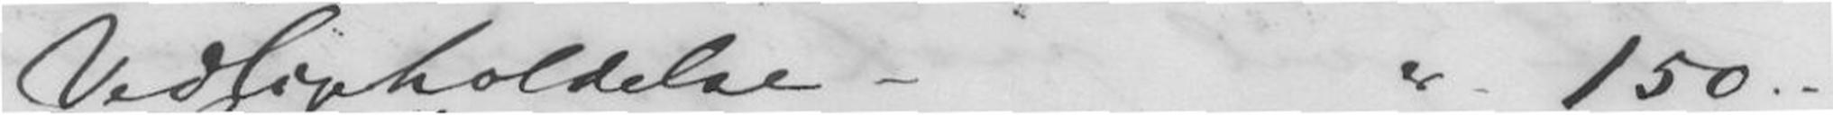Vedligeholdelse" 150.-
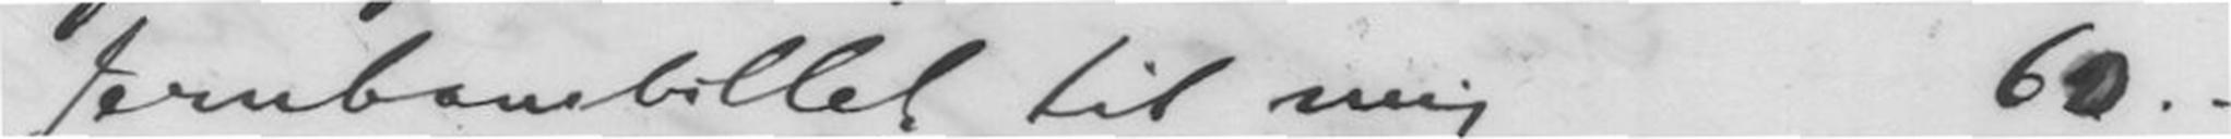Jernbanebillet til mig 60.-
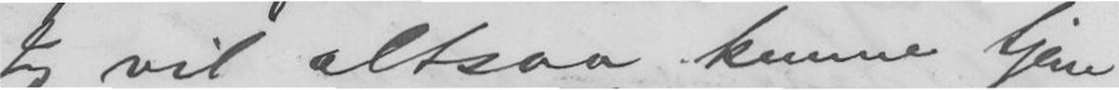Jeg vil altsaa kunne tjene
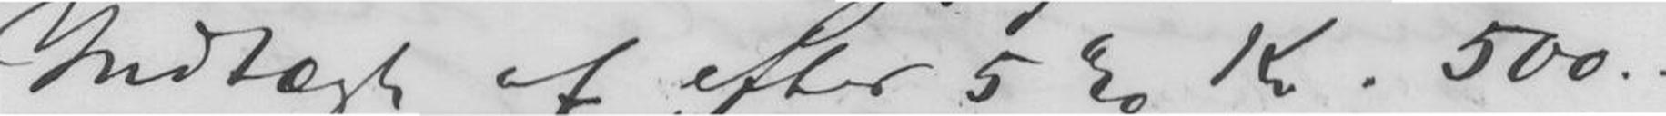Intægt af efter 5% Kr. 500.-
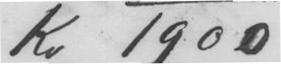Kr 1900.-
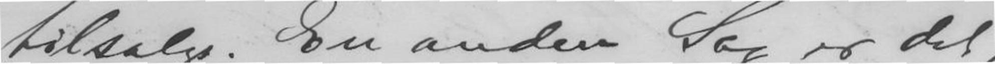tilsalgs. En anden Sag er det,
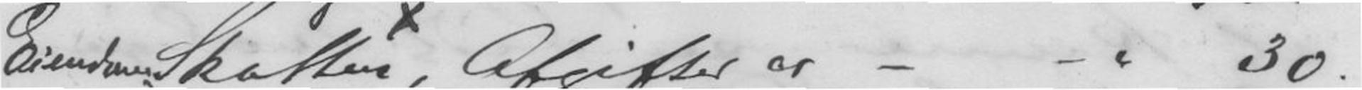EiendomsSkatterx, Afgifter ca." 30.-
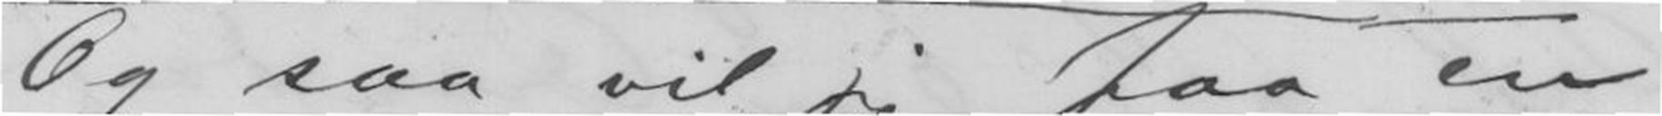Og saa vil jeg faa en
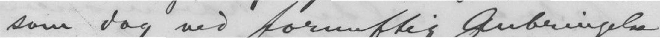som dog ved fornuftig Anbringelse
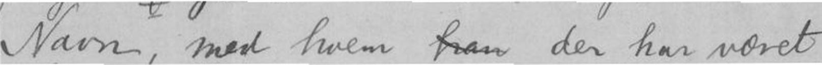Navn, med hvem han der har været
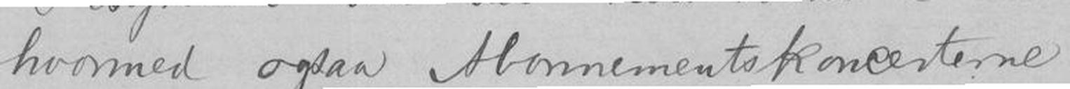hvormed ogsaa Abonnementskoncerterne
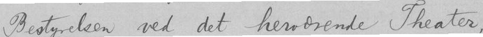Bestyrelsen ved det herværende Theater,
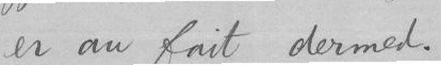er au fait dermed.-
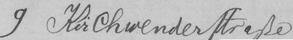9 Kirchwenderstraße
